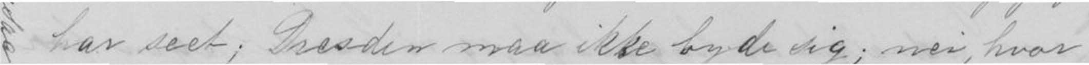har seet; Dresden maa ikke byde sig; men, hvor
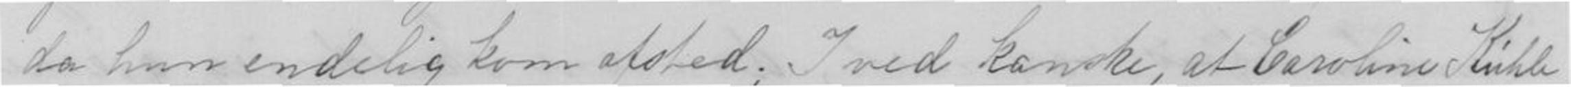da hun endelig kom afsted; I ved kanske, at Caroline Kuhb
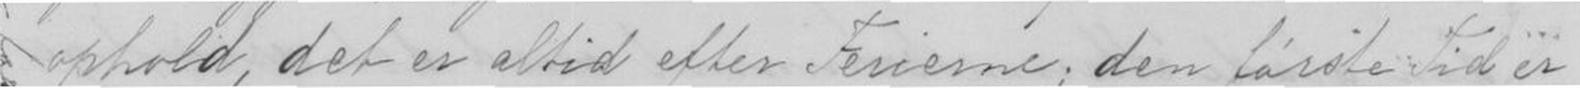ophold, det er altid efter Feriene; den første Tid er
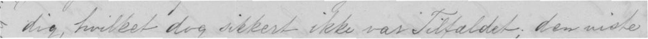dig, hvilket dog sikkert ikke var Tilfældet, den viste
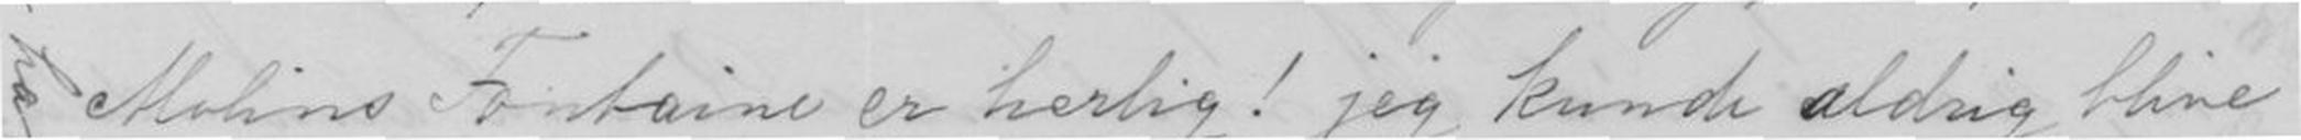Molins Fontaine er herlig! jeg kunde aldri blive
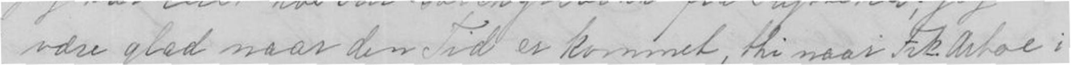være glad naar den Tid er kommet, thi naar Frk.Arboe i
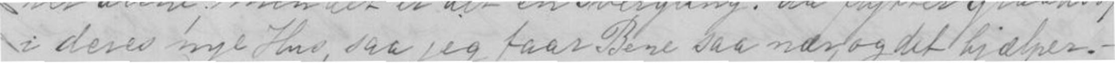i deres nye Hus, saa jeg faar Bere saa nær, og dit hjælper.-
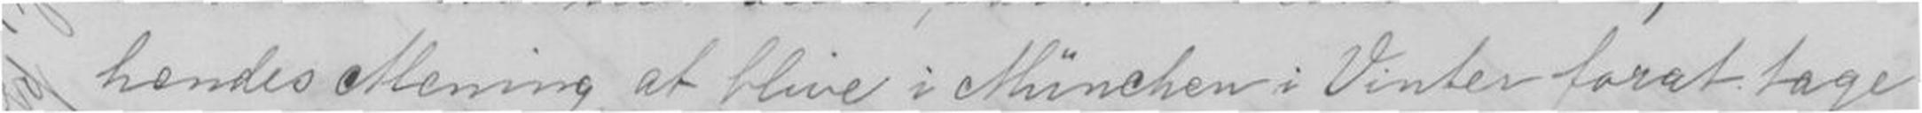hendes Mening at blive i Munchen i Vinter forat tage
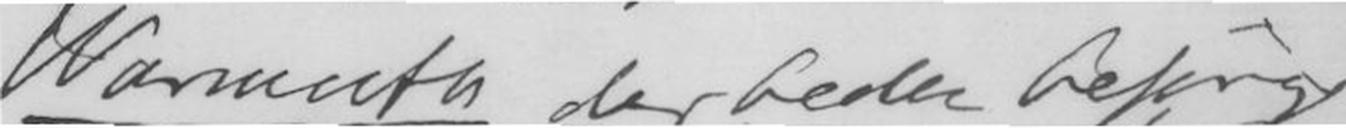Warmuth der beder besørge
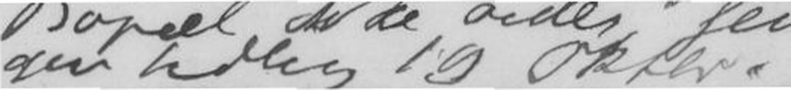gen tidlig 19 Oktbr.
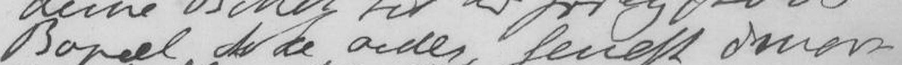Bopel til de vides senest imor-
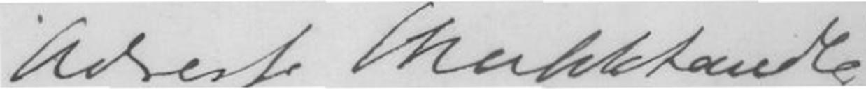Adresse Musikhandler
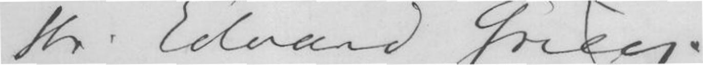Hr. Edvard Grieg.
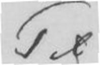Til
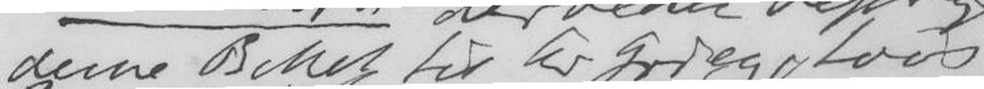denne Besked til hr. Grieg, hvis
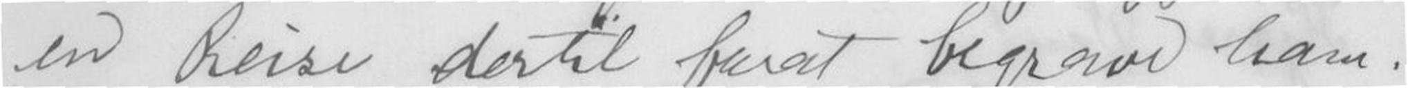en Reise dertil forat begrave ham.
